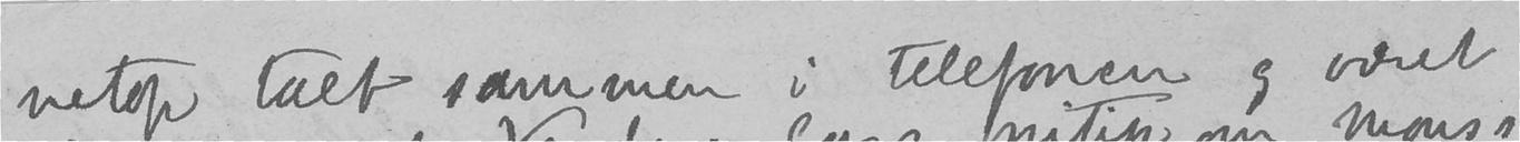netop talt sammen i telefonen og været
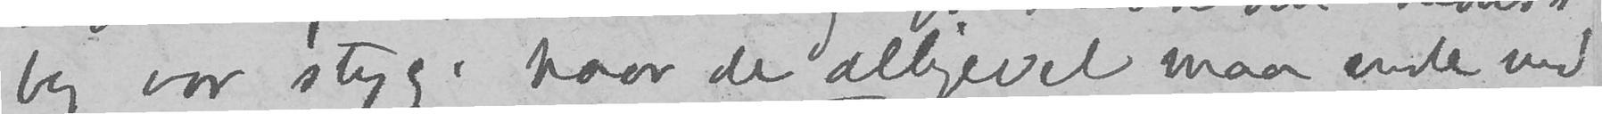bog var styg, naar de alligevel maa ende med
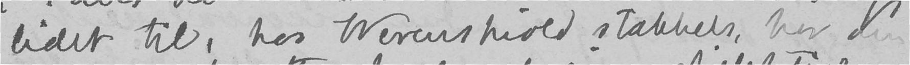lidet til, hos Werenskiold stakkels, har den
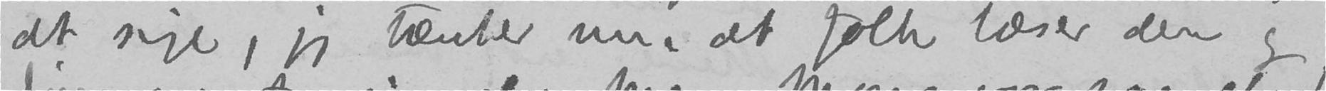at sige, jeg tænker nu, at folk læser den og
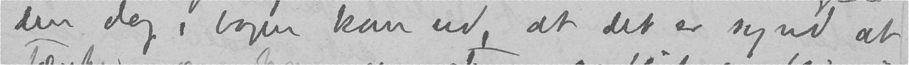den dag, bogen kom ud, at det er synd at
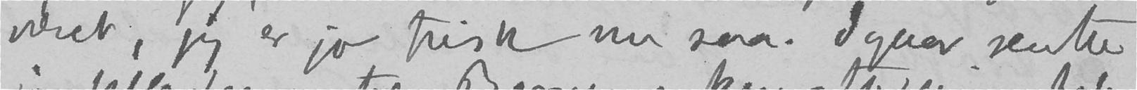været, jeg er jo frisk nu saa. Igaar sendte
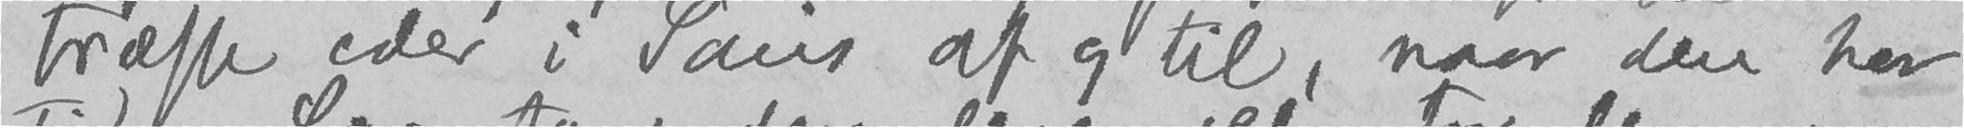træffe eder i Paris af og til, naar dere har
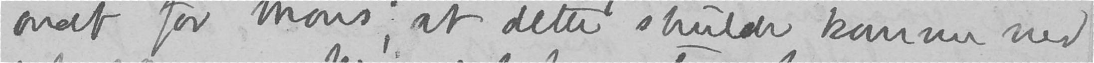ondt for Mons at dette skulde komme med
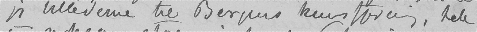jeg billederne til Bergens kunstforening, hele
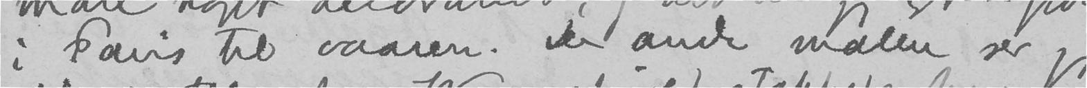i Paris til vaaren. De andre malere ser jeg
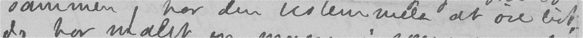sammen, har den bestemmelse at øve lidt.
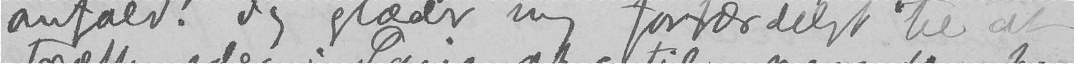anfald. Jeg glæder mig forfærdeligt til at
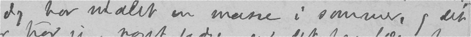Jeg har malet en masse i sommer, og det
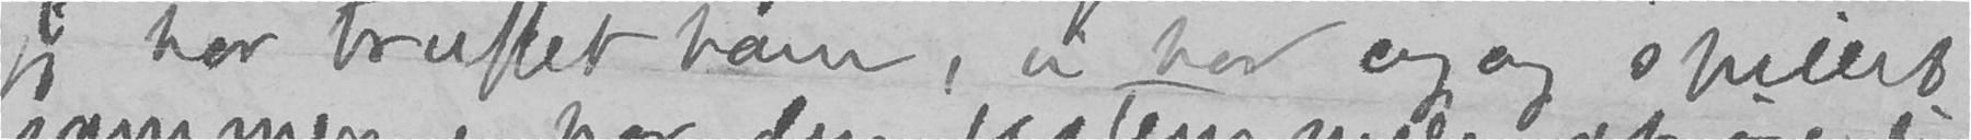jeg har truffet ham, vi har endog spillet
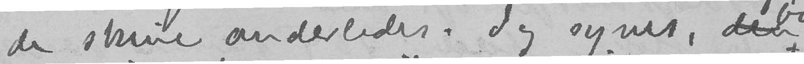de skrive anderledes. Jeg synes, den
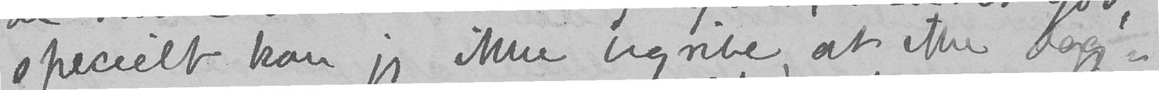specielt kan jeg ikke begribe, at ikke dag-
